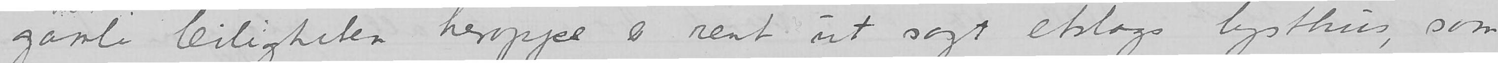gamle leiligheten heroppe er rent ut sagt etslags lysthus, som
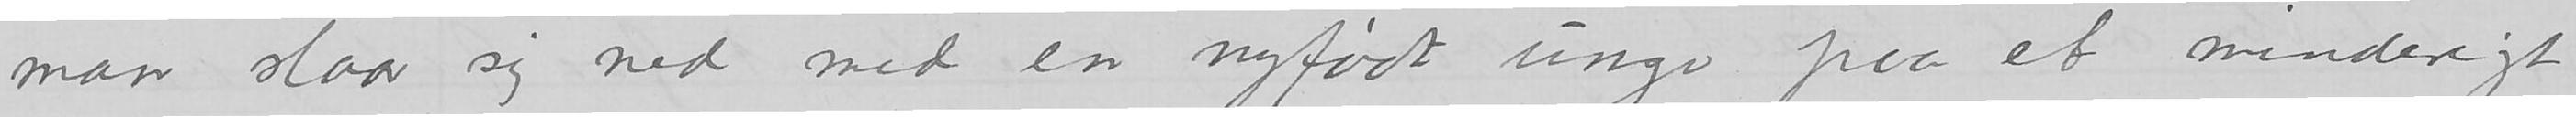man slaar sig ned med en nyfødt unge paa et minderigt
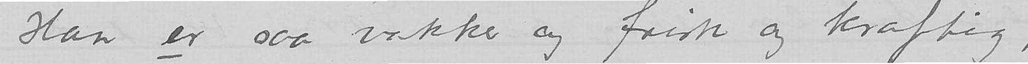Han er saa vakker og frisk og kraftig,
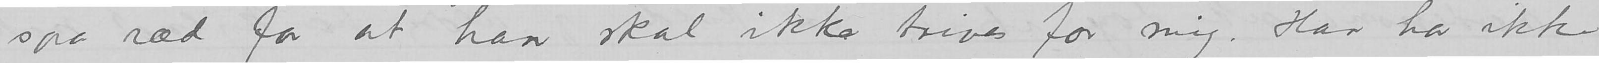saa ræd for at han skal ikke trives for mig. Han har ikke
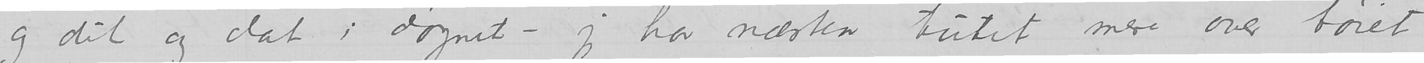og dit og dat i døgnet – jeg har næsten tutet mere over tøiet
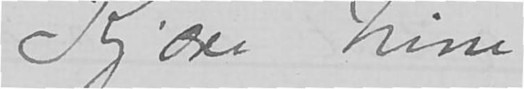Kjære Nini,
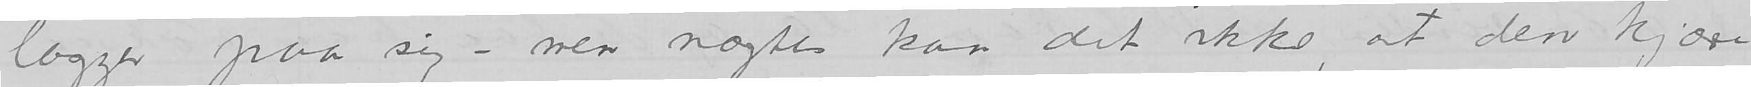lægger paa sig – men nægtes kan det ikke, at den kjære
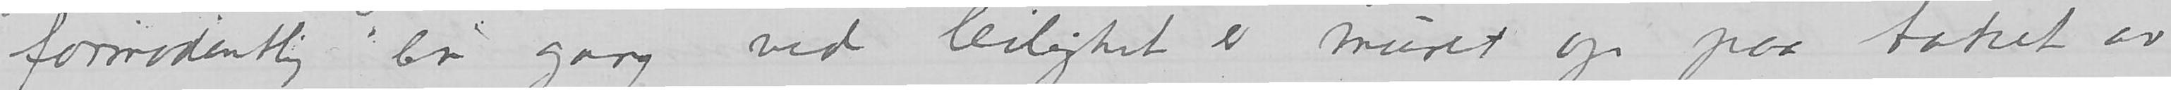formodentlig en gang ved leilighet er muret op paa taket av
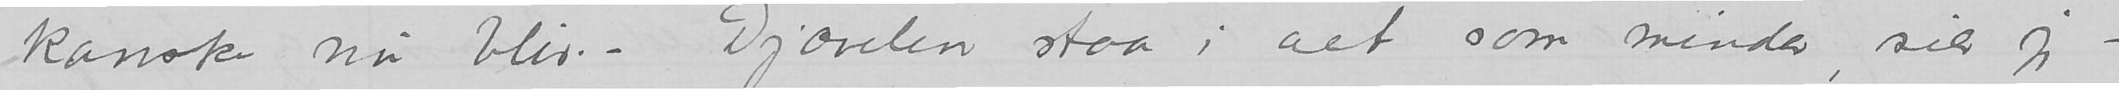kanske nu blir. – Djævelen staar i alt som minder, sier jeg –
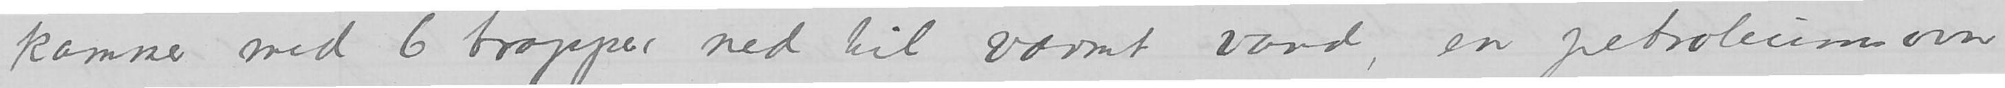kammer med 6 trapper ned til varmt vand, en petroleumsovn
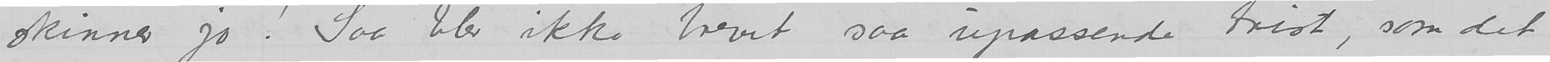skinner jo! Saa blev ikke brevet saa upassende trist, som det
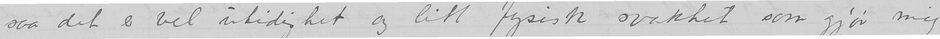saa det er vel utidighet og litt fysisk svakhet som gjør mig
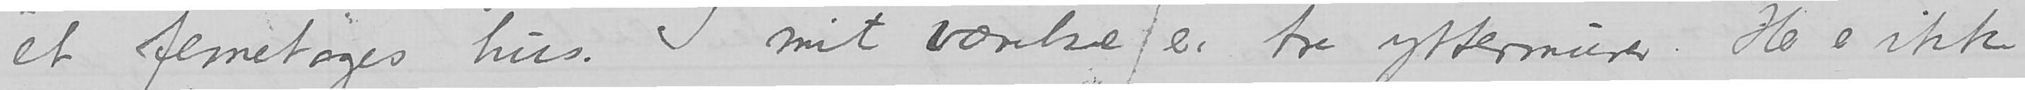et femetages hus. I mit værelse er tre yttermurer. Her er ikke
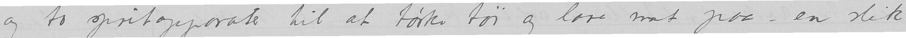og to spritapparater til at tørke tøi og lave mat paa – en slik
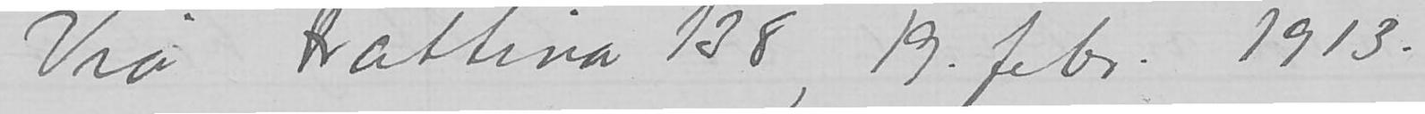Via Frattina 138, 19. febr. 1913.
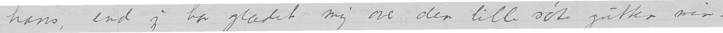hans, end jeg har glædet mig over den lille søte gutten min.
